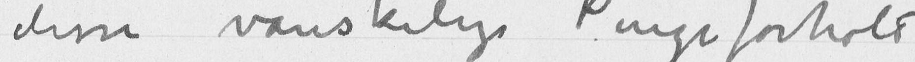disse vanskelige Pengeforhold
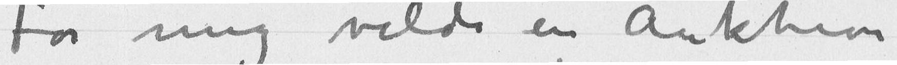For mig vilde en Auktion
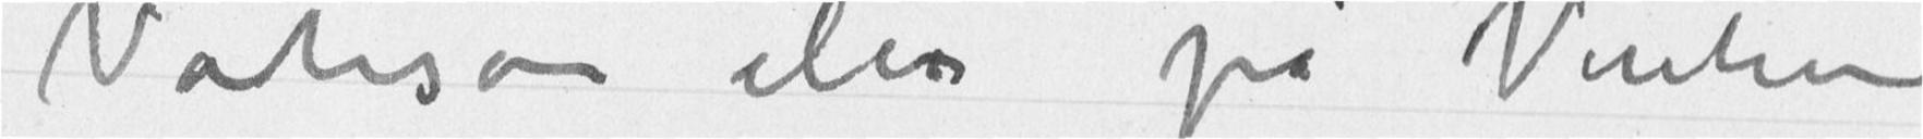Vasteson eller på Vinteren
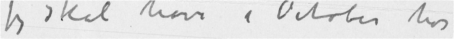jeg skal have i October hos
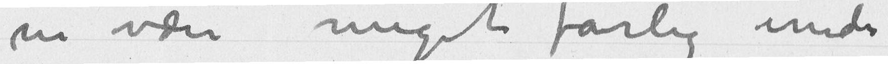nu være meget farlig under
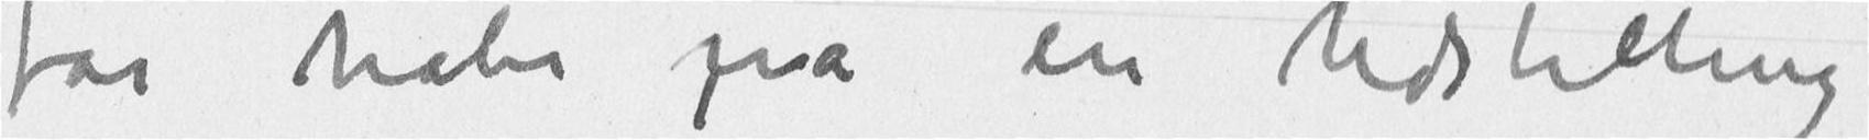får håbe på en Udstilling
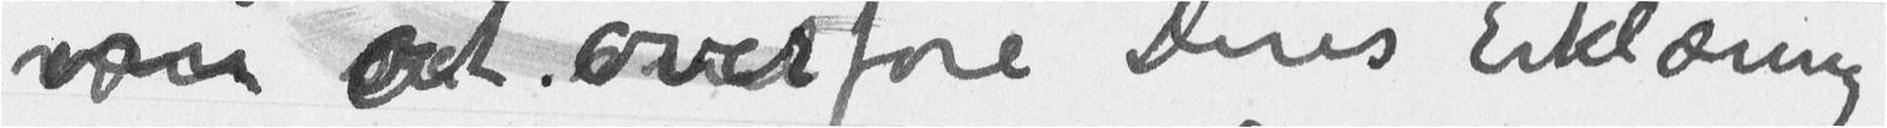væreom at overføre Deres Erklæring
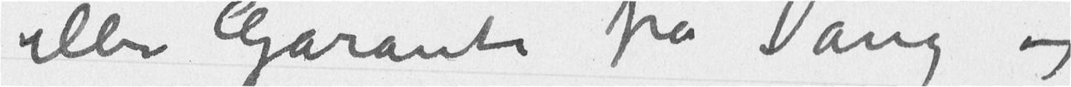eller Garanti fra Vang og
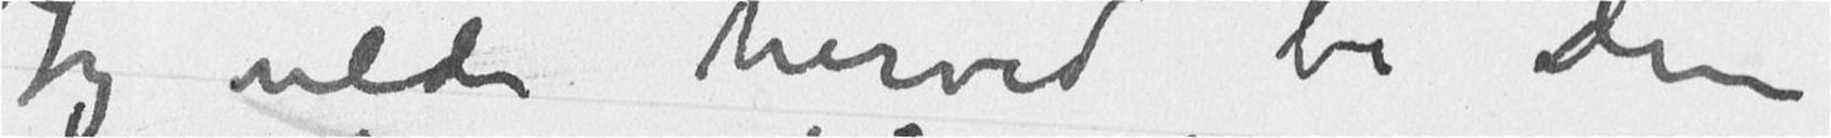Jeg vilde herved be Dem
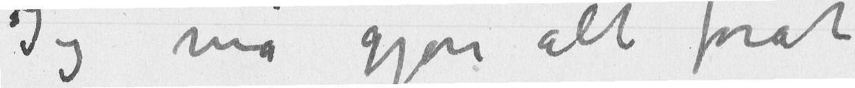Jeg må gjøre alt forat
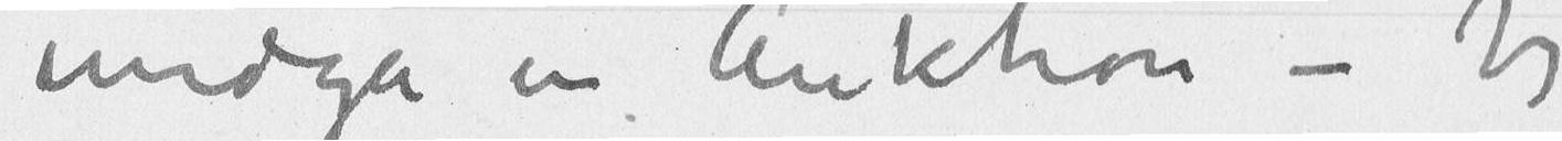undgå en Auktion – Jeg
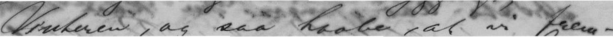Vinteren, og saa haabe, at vi frem-
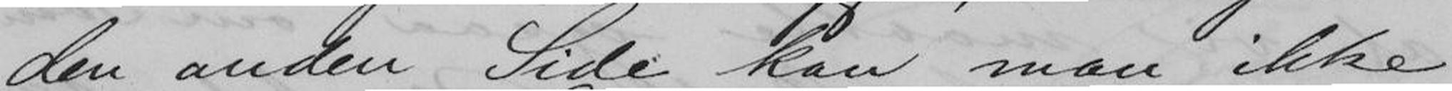den anden Side kan man ikke
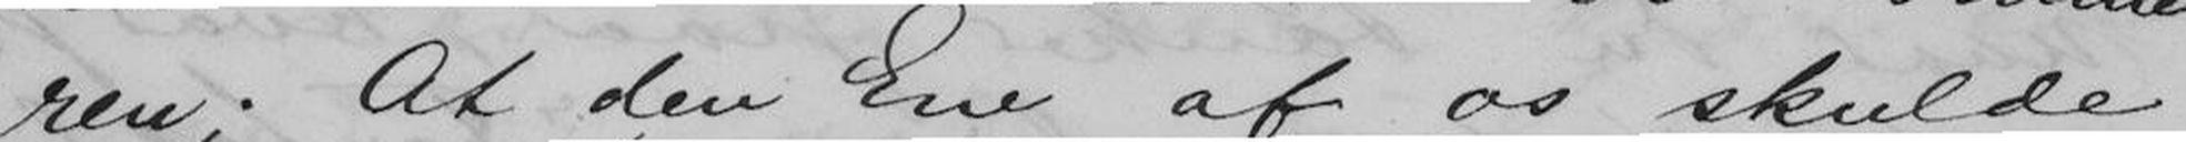ren. At den Ene af os skulde
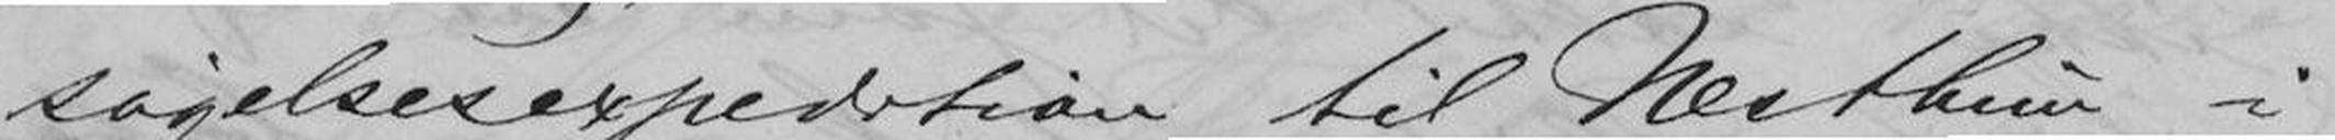søgelsexpedition til Nesthun i
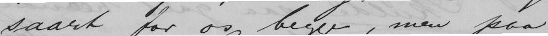saart for os begge, men paa
